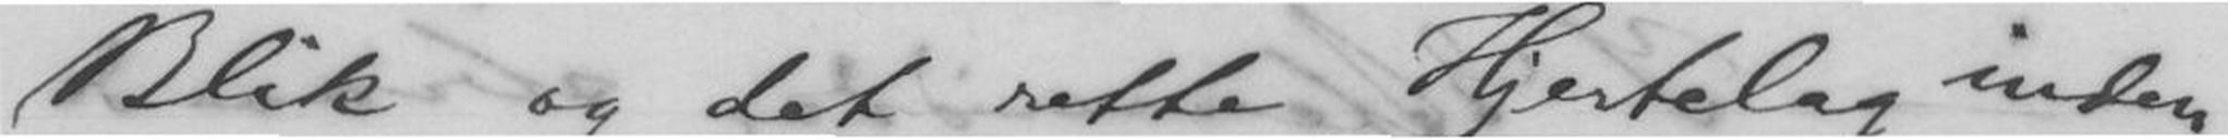Blik og det rette Hjertelag inden-
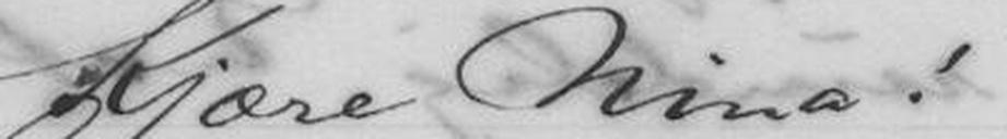Kjære Nina!
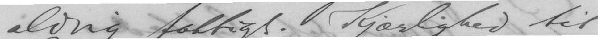aldrig fattigt. Kjærlighed til
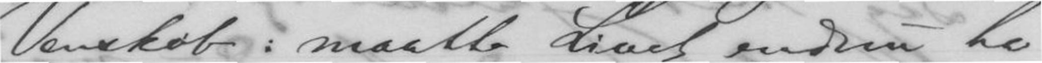Venskab: maatte Livet endnu ha
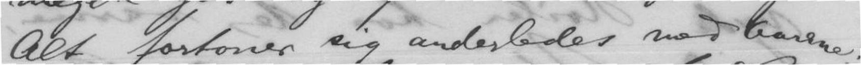Alt fortoner sig anderledes med Aarene:
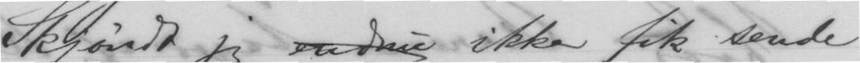Skjøndt jeg endnu ikke fik sende
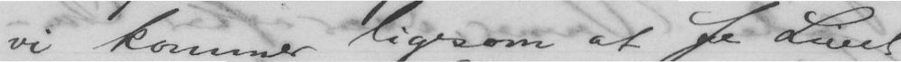vi kommer ligesom at se Livet
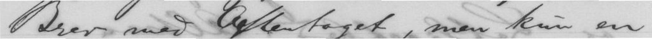Brev med Aftentoget, men kun en
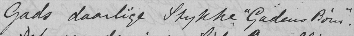Gads daarlige Stykke "Gadens Børn".
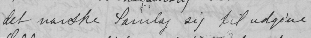det norske Samlag sig til udgive
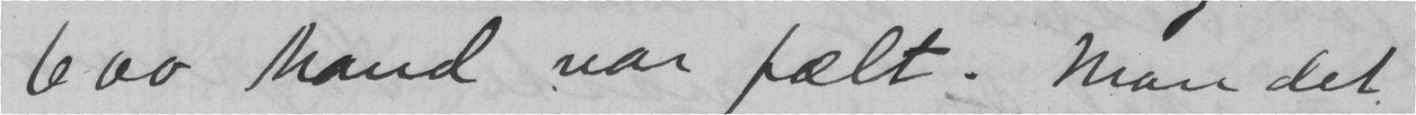600 Mand var fælt. Mon det
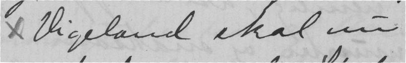x Vigeland skal nu
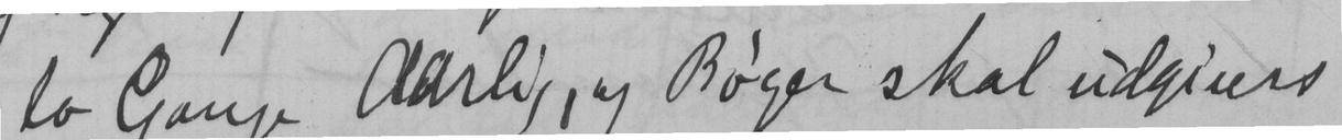to Ganpe Aarlig, og Bøger skal udgivers
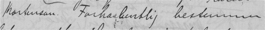Mortenson. Forhaabentlig bestemmer
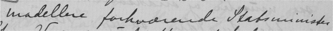modellere forhværende Statsmimister
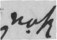nok
